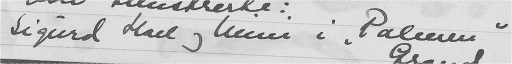Sigurd Hoel og Nini i "Palenen
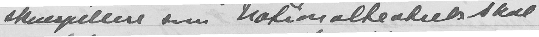skuespillere som Nationaltheatrets skal
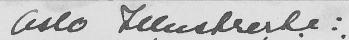Oslo Hristrerte:
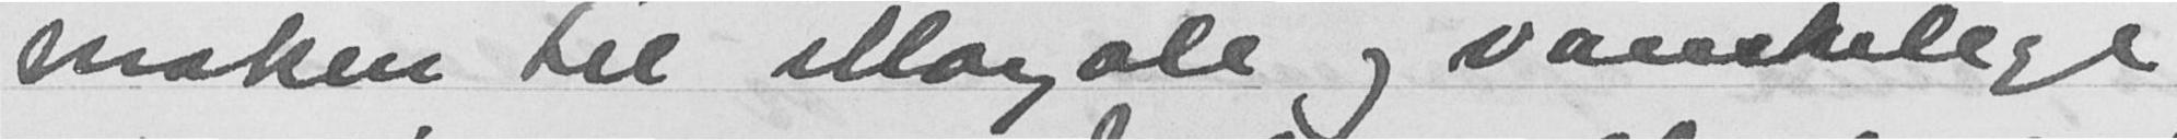maken til illoyale og vanskelige
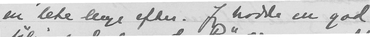en lete lenge efter. Jeg hadde en god
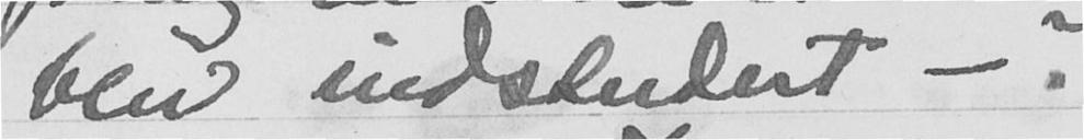blev indstudert - .
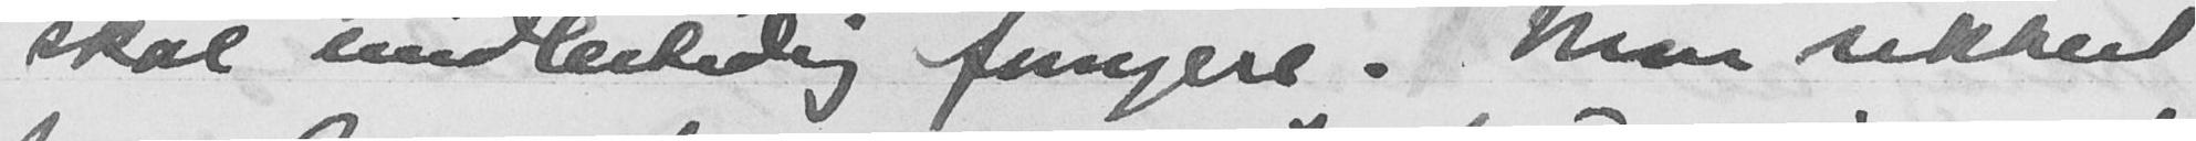skal imidlertid fungere. Men sikkert
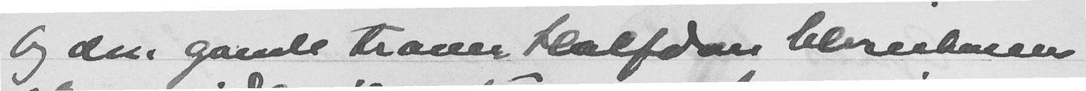Og den gamle traver Halfdan Christensen
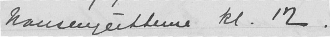Nansengutterne kl. 12.
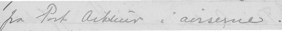fra Port Arthur i aviserne.
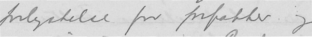forlystelse for forfatter og
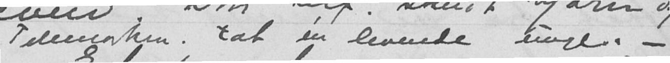Telmarken. tat en levende unge. -
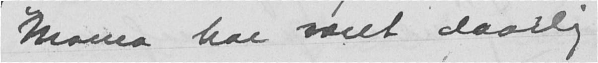Mama har været daarlig
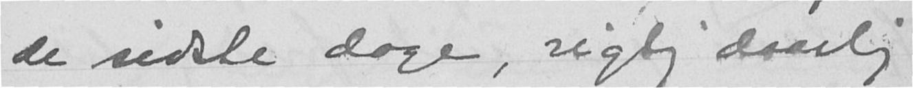de sidste dage, rigtig daarlig
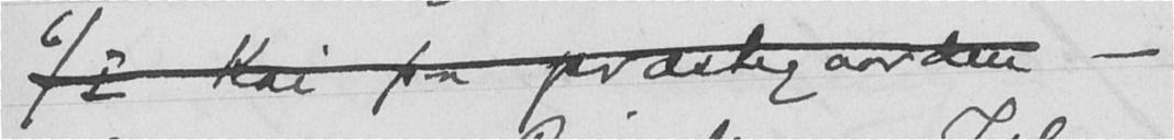6/I Kai paa præstegaarden -
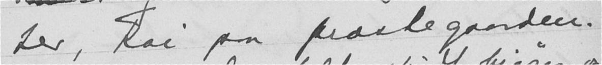her, Kai paa præstegaarden.
